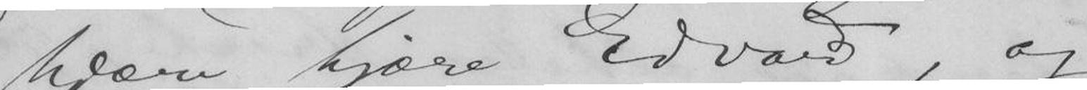kjære kjære Edvard, og
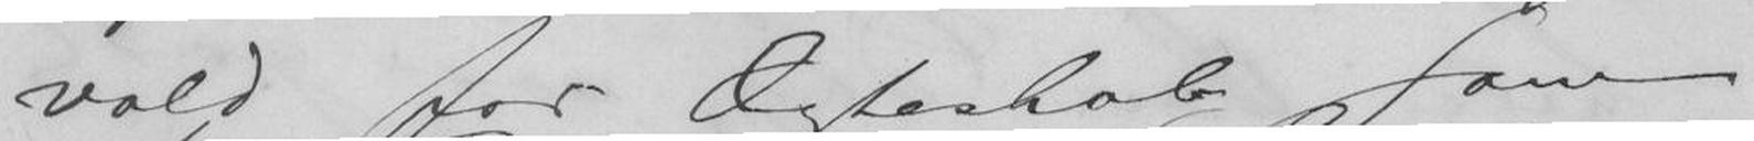vold for Ægteskab som
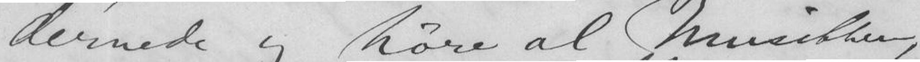dernede og høre al Musikken,
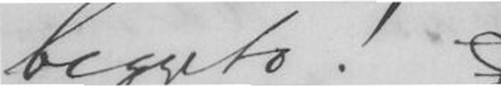beggeto!
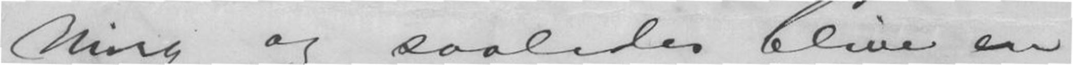ning og saaledes blive en
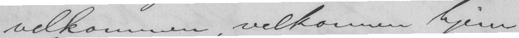velkommen, velkommen hjem
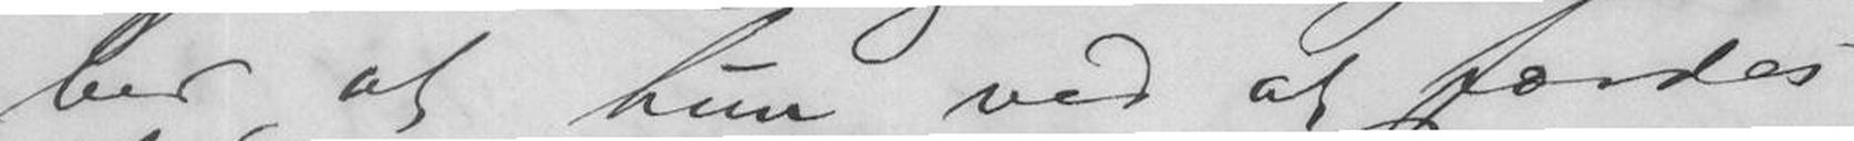ber, at hun ved at færdes
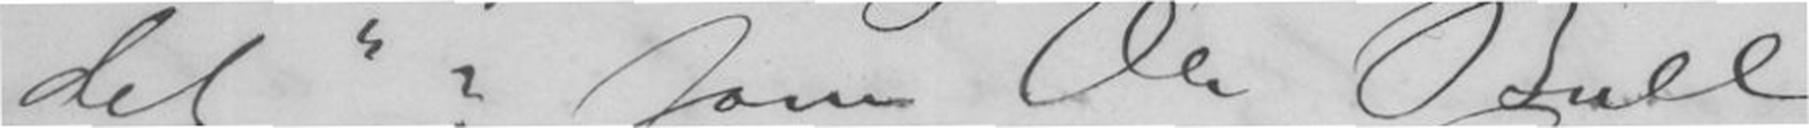det"? som Ole Bull
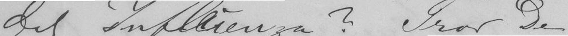det Influenza? "Tror De
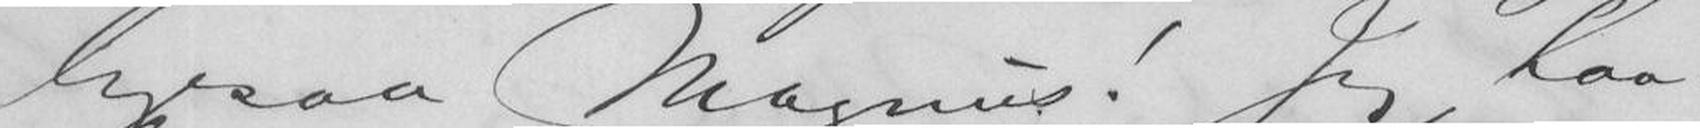ligesaa Magnus! Jeg haa-
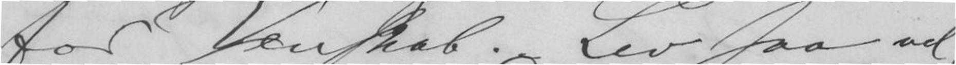for Venskab. Lev saa vel,
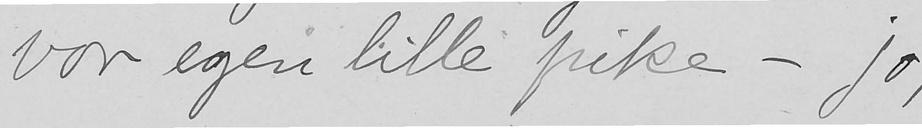vor egen lille pike - jo,
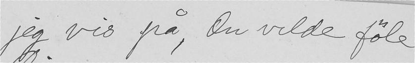jeg vis på, Du vilde føle
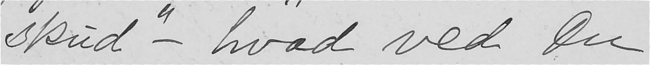skud" - hvad ved Du
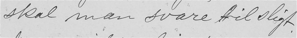skal man svare til sligt.
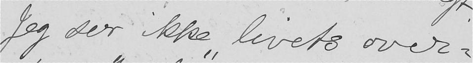Jeg ser ikke "livets over-
